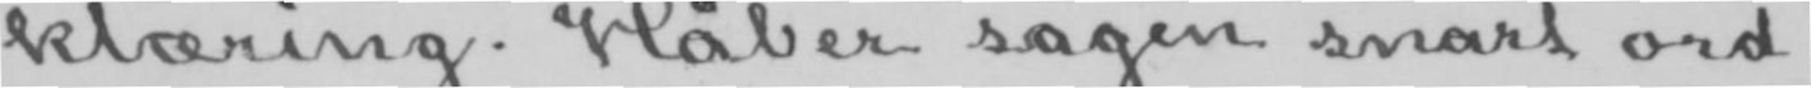klæring. Håber sagen snart ord-
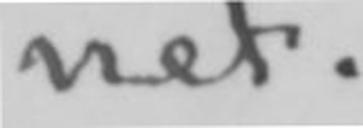net.
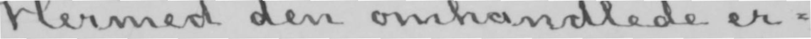Hermed den omhandlede er-
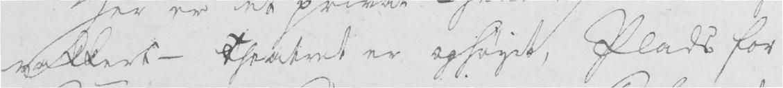vakkert - Theatret er ophøyet, Plads for
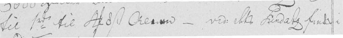til 1 rd til 4 f 8 s Aleen - vid. dets Fundatz findes i
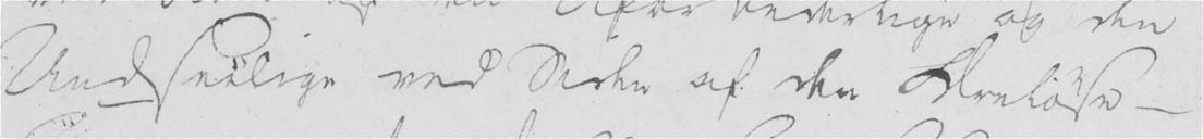Undseelige ved Siden af den Æreløse -
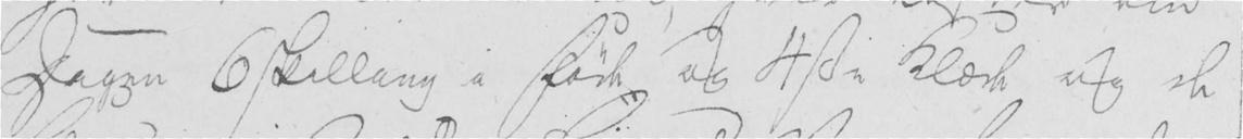Dagen 6 skilling i Føde og 4 sr Klæde og de
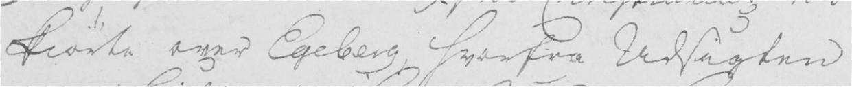kiørte over Egeberg, hvorfra Udsigten
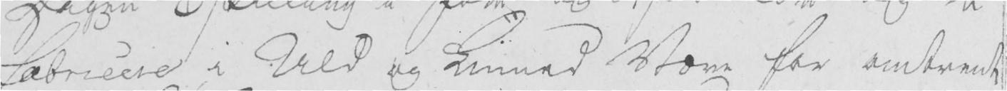fabricere i Uld og Linned Vare for omtrent
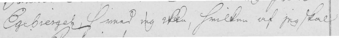Egeberget, veed jeg ikke, hvilken af jeg skal
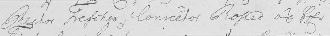Rector Treschow, Conrector Rosted og Hr
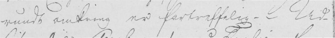rundt omkring er fortreffelig - - Ud-
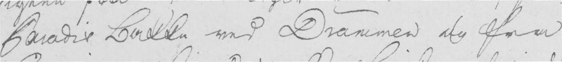Paradis Bakke ved Drammen og fra
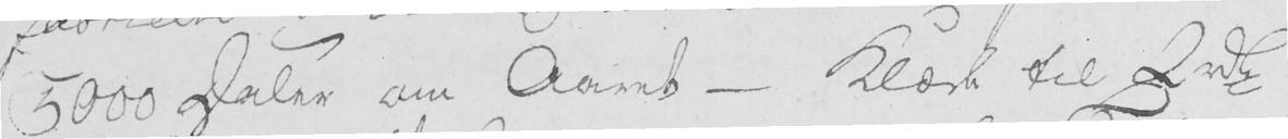5000 Daler om Aaret - Klæde til 2 rde
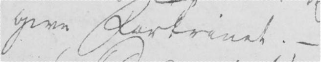give Fortrinet. -
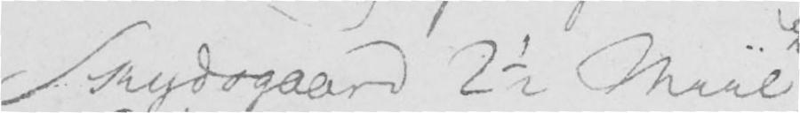Rydsgaard 2 ½ Miil
